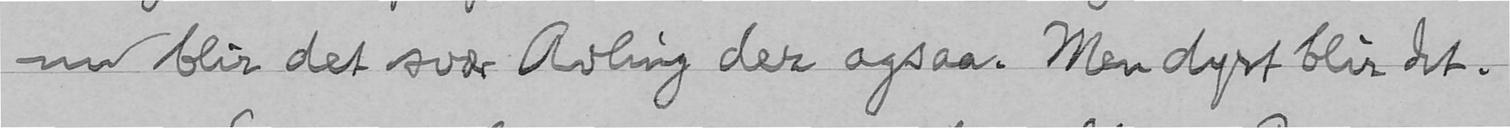nu blir det svær Avling der ogsaa. Men dyrt blir det.
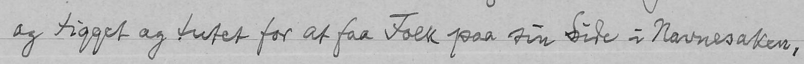og tigget og tutet for at faa Folk paa sin Side i Navnesaken,
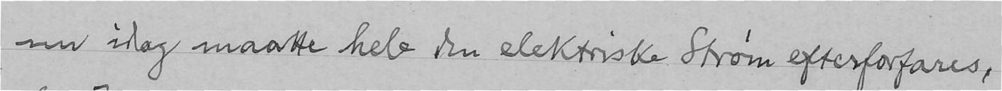nu idag maatte hele den elektriske Strøm efterforfares,
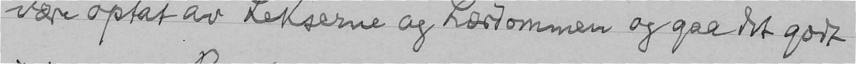være optat av Lekserne og Lærdommen og gaa det godt
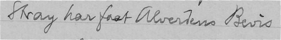Stray har faat Alverdens Bevis
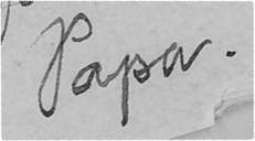Papa.
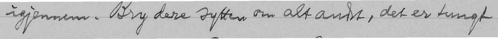igjennem. Bry dere sytten om alt andet, det er tungt
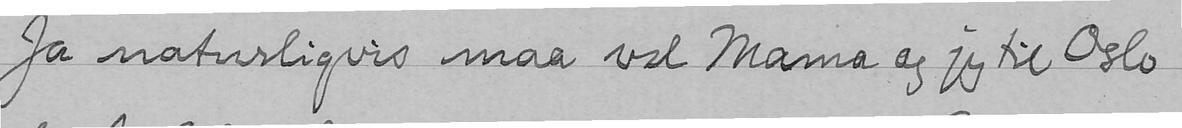Ja naturligvis maa vel Mama og jeg til Oslo
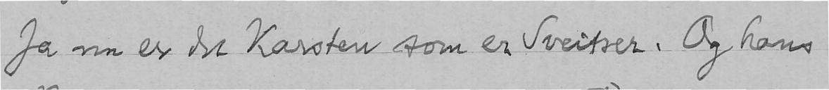Ja nu er det Karsten som er Sveitser. Og hans
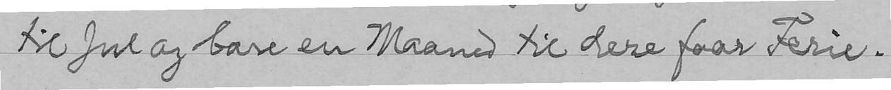til Jul og bare en Maaned til dere faar Ferie.
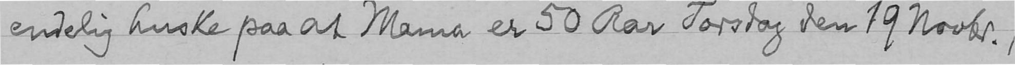endelig huske paa at Mama er 50 Aar Torsdag den 19 Novbr.,
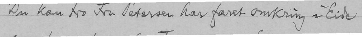Du kan tro Fru Petersen har faret omkring i Eide
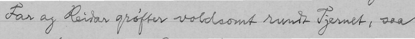Far og Reidar grøfter voldsomt rundt Tjernet, saa
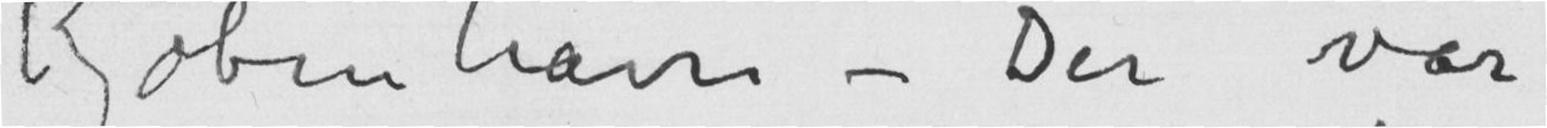Kjøbenhavn – Der var
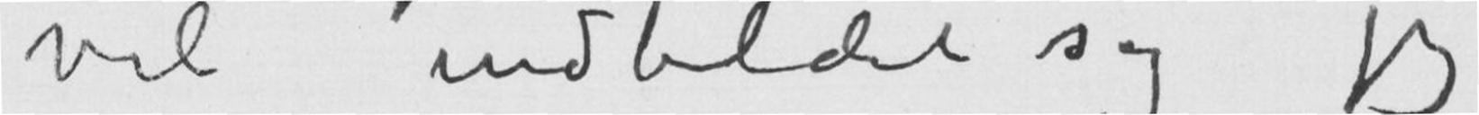vel indbildet sig jeg
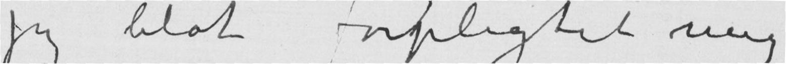jeg blot forpligtet mig
